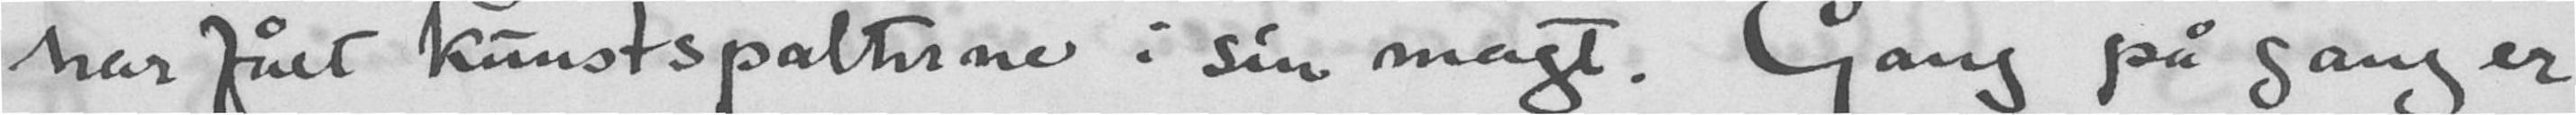har fået kunstspalterne i sin magt. Gang på gang er
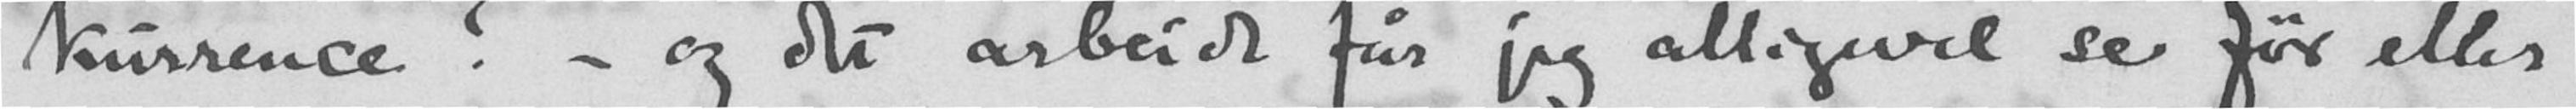kurrence? - og det arbeide får jeg alligevel se før eller
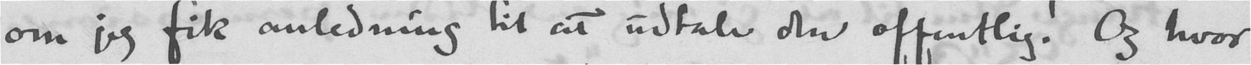om jeg fik anledning til at udtale den offentlig! Og hvor
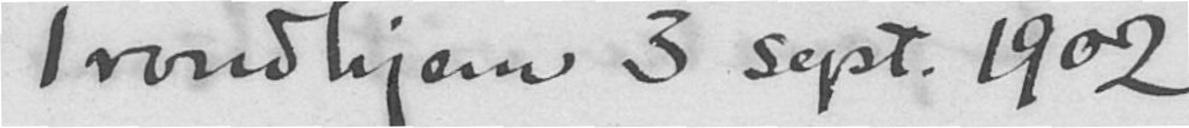Trondhjem 3 sept. 1902
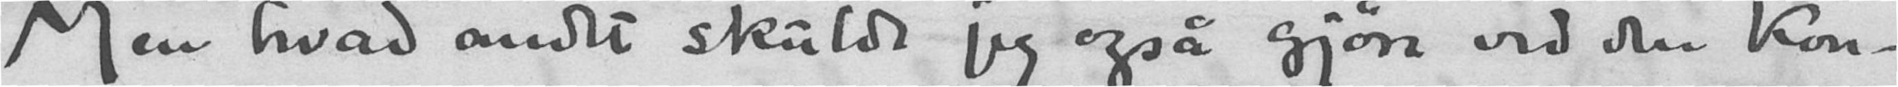Men hvad andet skulde jeg også gjøre ved den kon-
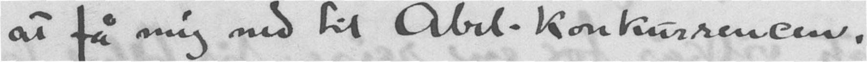at få mig ned til Abel-konkurrencen.
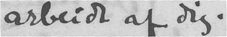arbeide af dig.
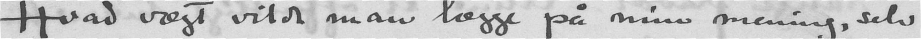Hvad vægt vilde man lægge på mine mening, selv
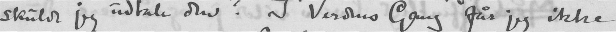skulde jeg udtale den? I "Verdens Gang" får jeg ikke
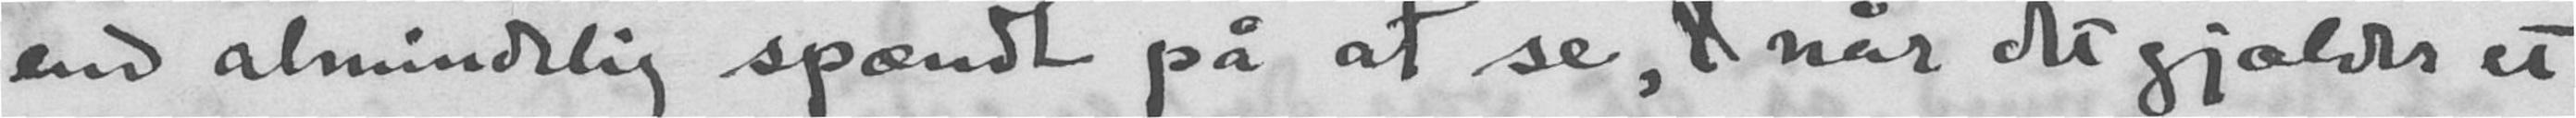end almindelig spændt på at se, når det gjælder et
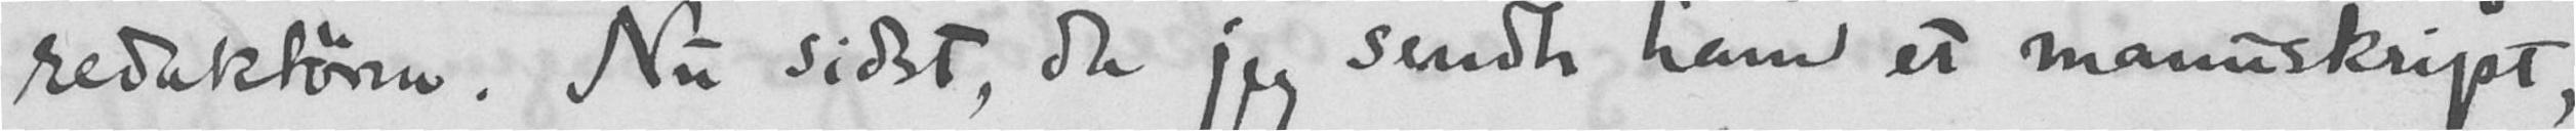redaktøren. Nu sidst, da jeg sendte ham et manuskript,
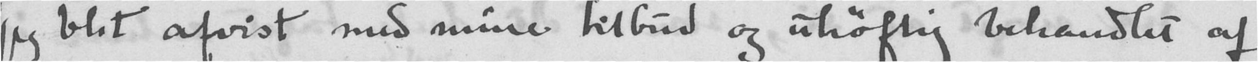jeg blit afvist med mine tilbud og uhøflig behandlet af
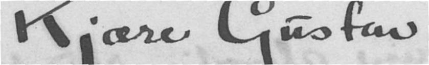Kjære Gustav
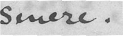senere.
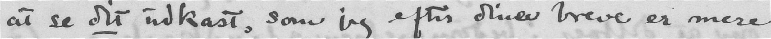at se dit udkast, som jeg efter dine breve er mere
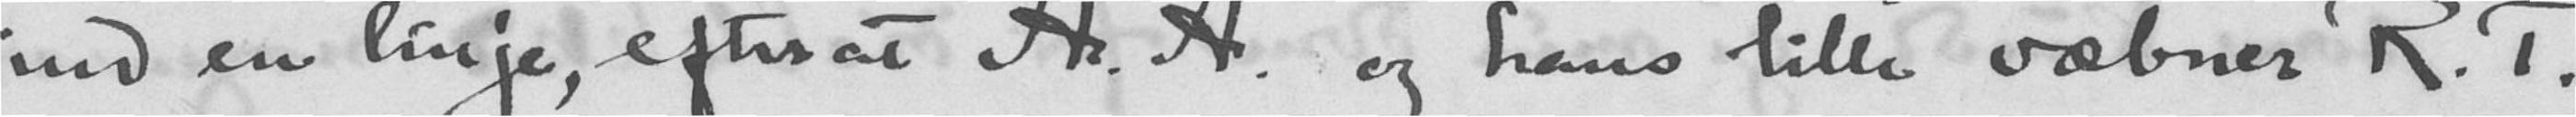ind en linje, efter at A.A. og hans lille væbner R.T.
